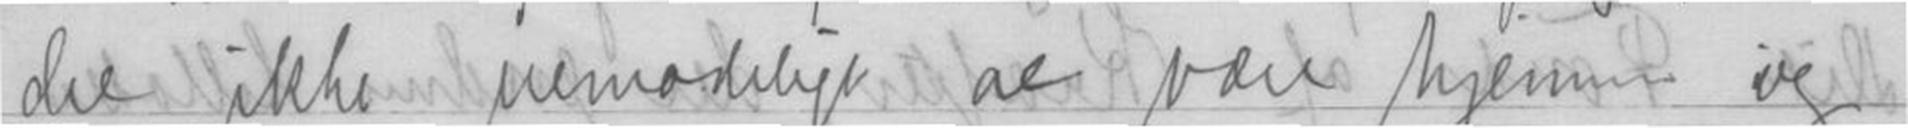det ikke vemodeligt at være hjemme og
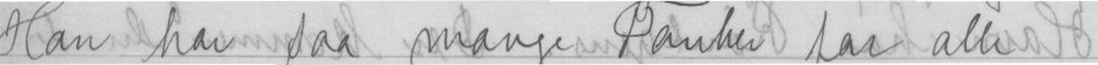Han har saa mange Tanker for alle
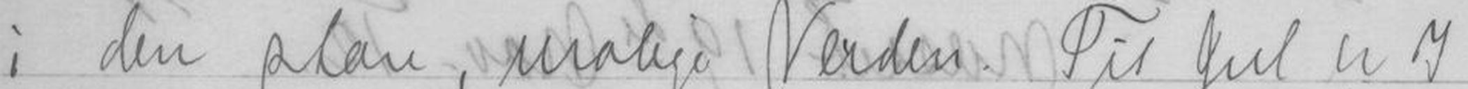i den store, urolige Verden. Til Jul er I
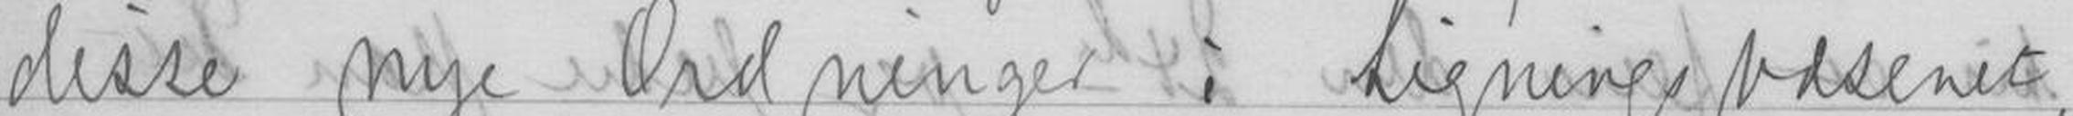disse nye Ordninger i Ligningsvæsenet,
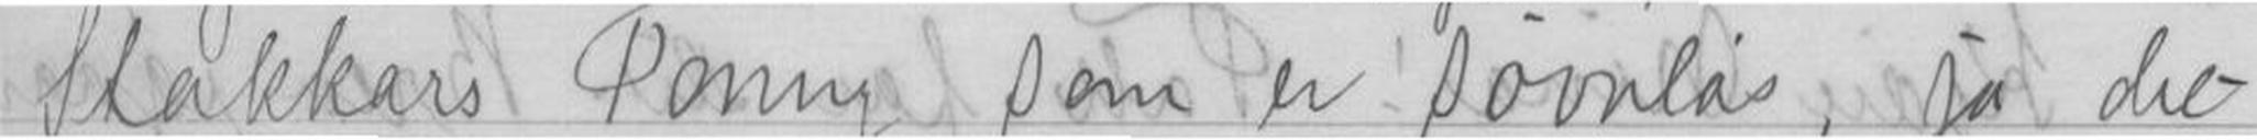Stakkars Tonny som er søvnløs, ja det
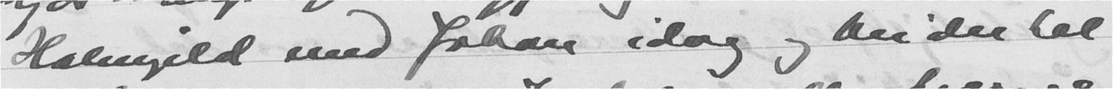Holmgild med Johan idag og bli der til
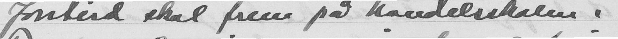Fonteid skal frem på handelsskolen i
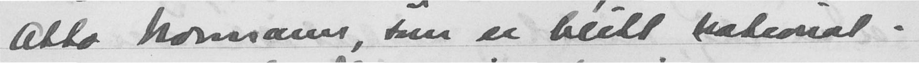Otto Normann, som er blitt national-
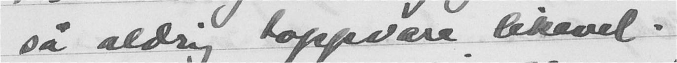så aldrig toppvære likevel.
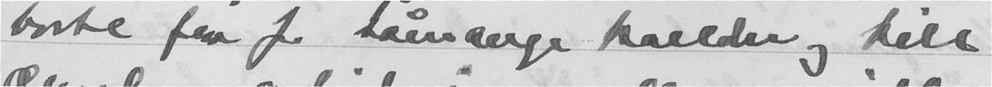borte fra J. såmange kvelder og till
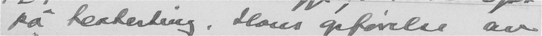pa teaterting. Hans opførelse av
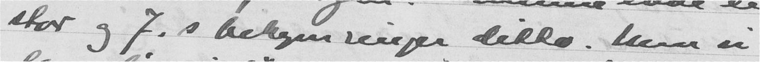stor og J.s bekymringer ditto. Men vi
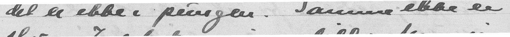det er ikke i pungen. Sammen ikke er
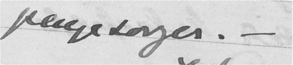pengesorger. -
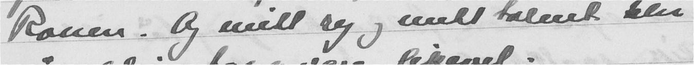Rouen. Og mitt ry og mitt talent blir
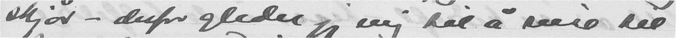skjør - derfor gleder jeg mig til å reise til
